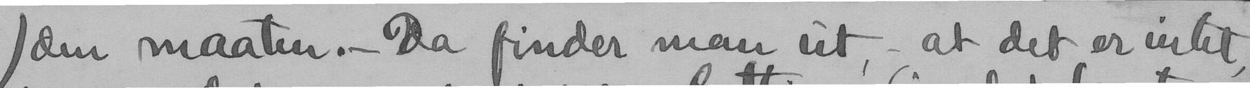den maaten. - Da finder man ut, - at det er intet,
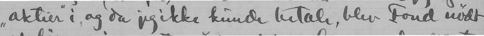"aktier" i og da jeg ikke kunde betale, blev Fond nødt
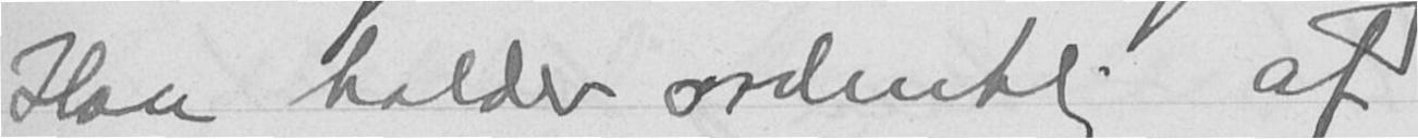Han holder ordentlig af
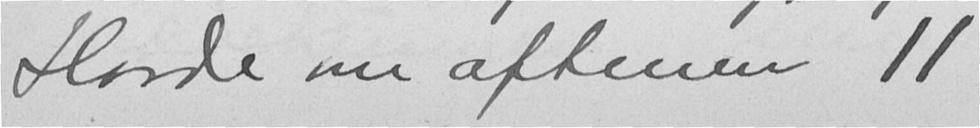Havde om aftenen 11
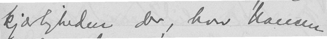kjærligheden der, hvor Nansen
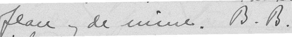Jean og de mine. B. B.
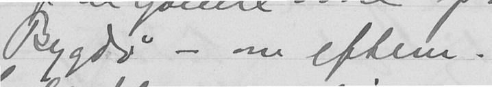Bygdø - om efterm.
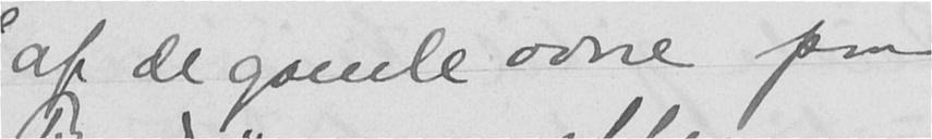af de gamle ovne paa
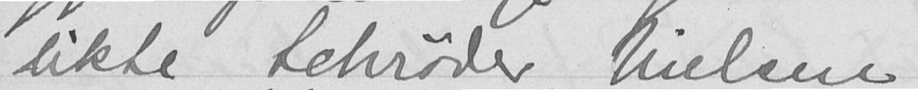likte Schrøder Nielsen
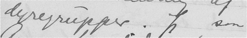dyregrupper. Jeg saa
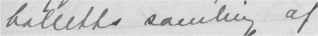Colletts samling af
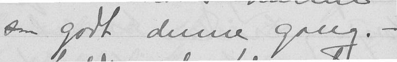saa godt denne gang. -
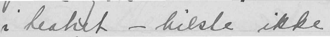i teatret - hilste ikke
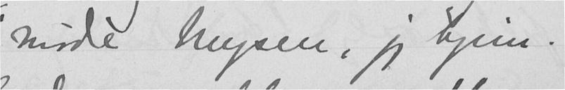møde Mysen, jeg hjem.
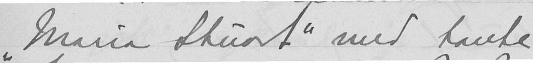"Maria Stuart" med tante
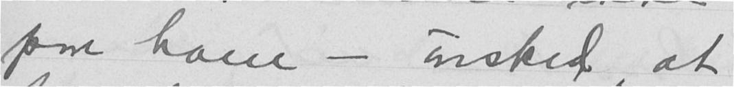paa ham - ønsked, at
